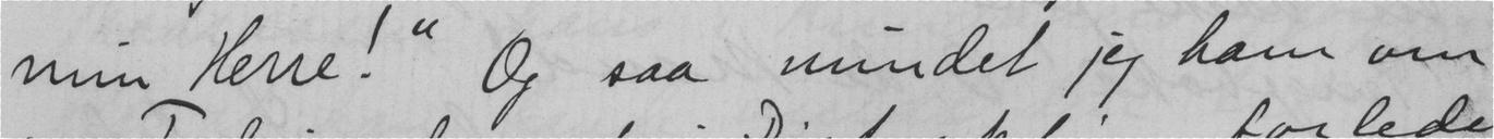min Herre!" Og saa mindet jeg ham om
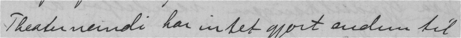Theater nemdi har intet gjort endnu til
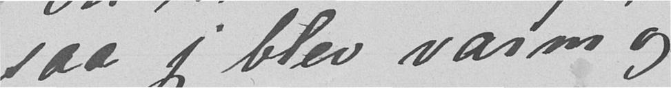saa jeg blev varm og
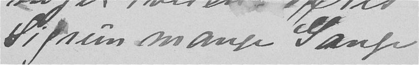Sigrun mange Gange
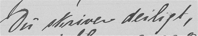Du skriver deiligt,
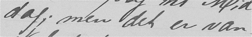dag; men det er van-
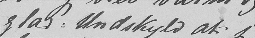glad. Undskyld at jeg
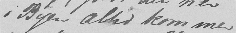i Byen altid kommer
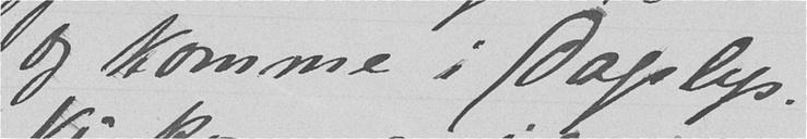og komme i Dagslys.
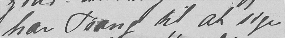har Trang til at sige
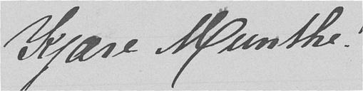Kjære Munthe.
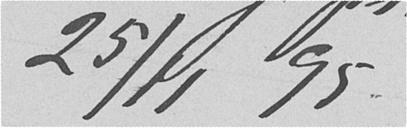25/11 95.
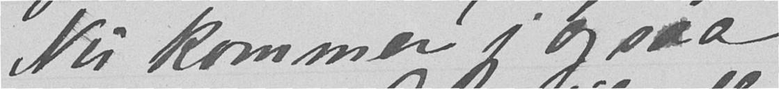Nu kommer jeg ogsaa
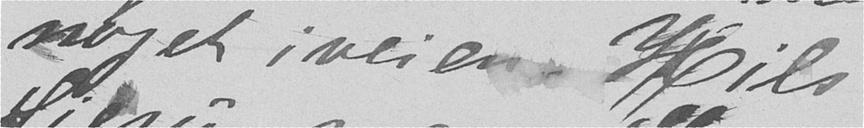noget iveien. Hils
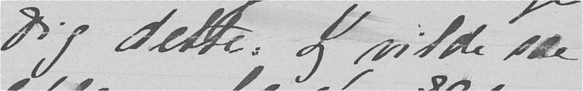dig dette. Jeg vilde saa
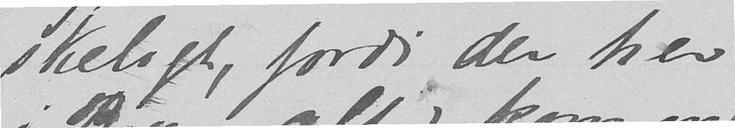skeligt, fordi der her
